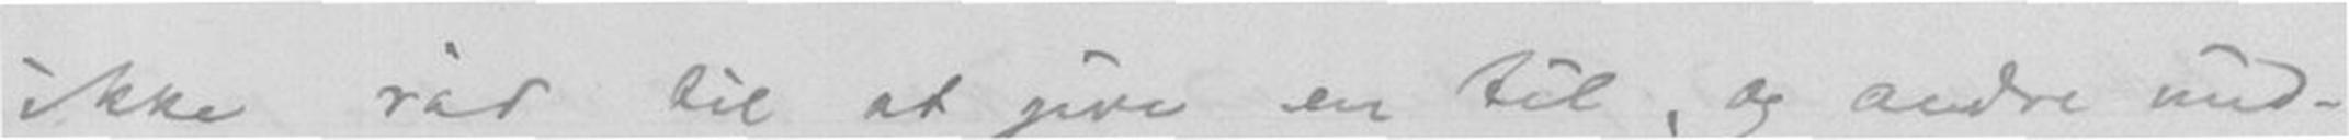ikke råd til at give en til, og andre mid-
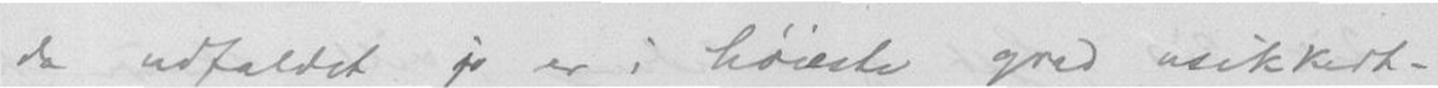da udfaldet jo er i høiste grad usikkert -
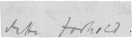dette forhold.
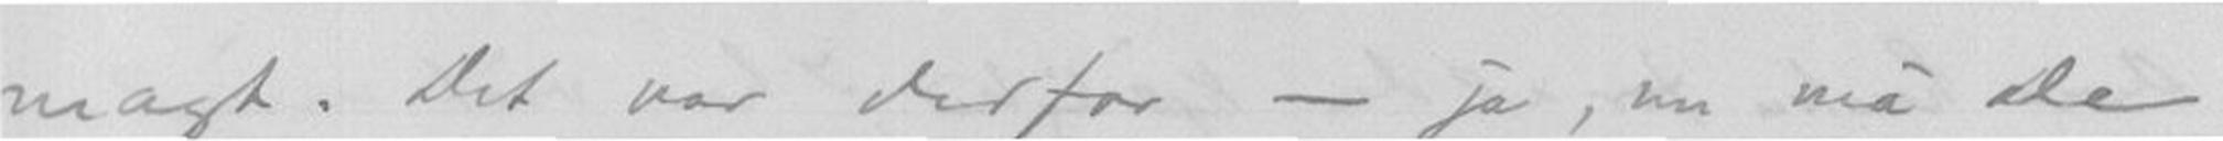magt. Det var derfor - ja, nu må De
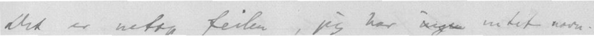Det er netop feilen, jeg har ingen intet navn.
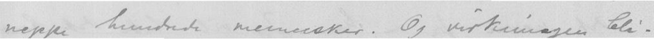neppe hundrede mennesker. Og virkningen bli-
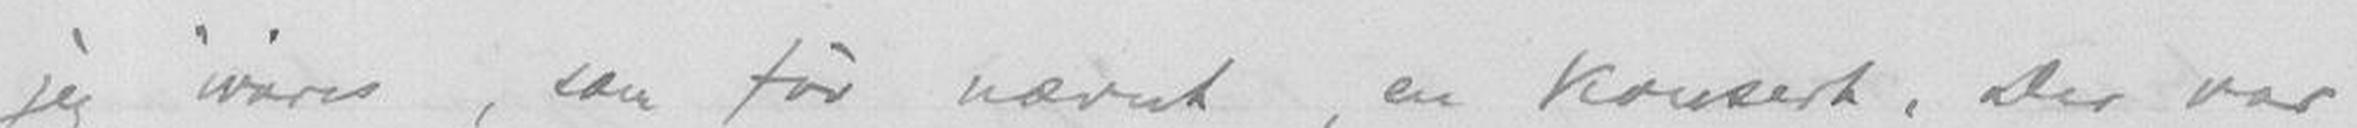jeg ivåres, som før nævnt, en konsert. Der var
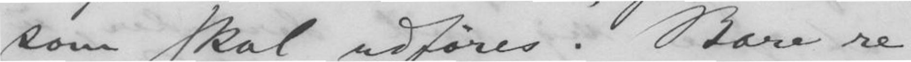som skal udføres. Bare re-
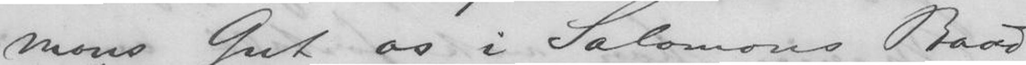mons Gut os i Salomons Baad
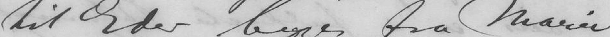til Eder begge fra Marie
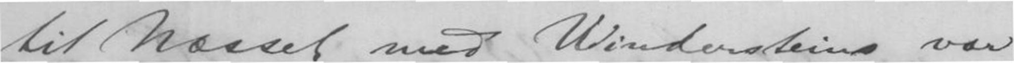til Næsset med Winderstein var
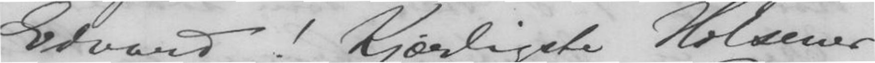Edvard! Kjærligste Hilsener
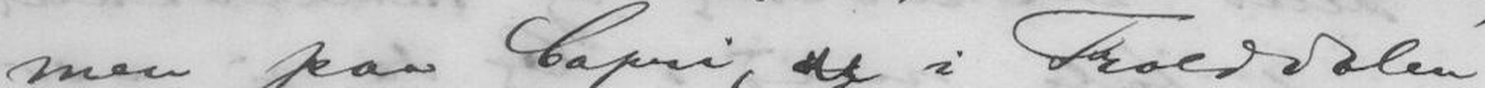men paa Capri, og i Trolddalen
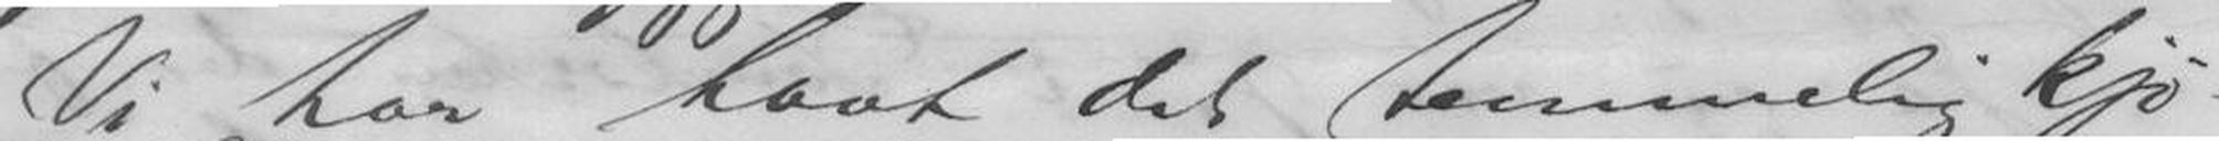Vi har havt det temmelig kjø-
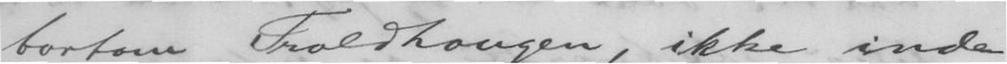bortom Troldhougen, ikke inde,
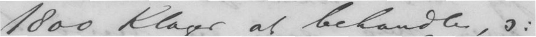1800 Klager at behandle, ):
